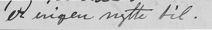er ingen nytte til.
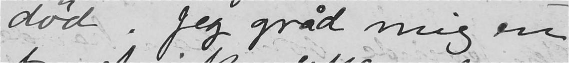død. Jeg gråd mig en
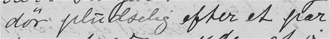dør plutselig efter et par
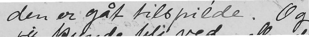den er gåt tilspilde. Og
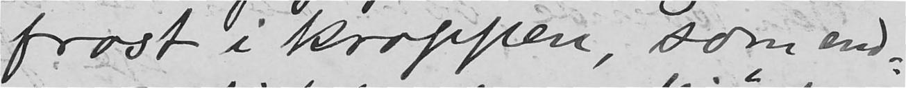frost i kroppen, som end-
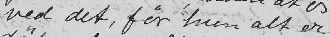ved det, før hun alt er
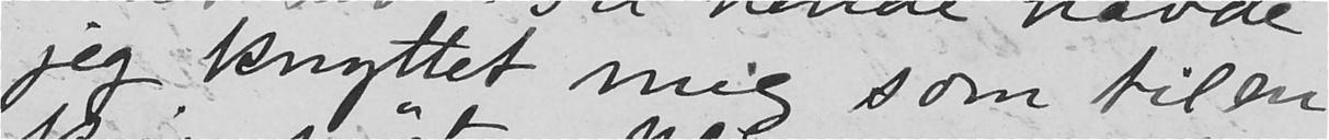jeg knyttet mig som til en
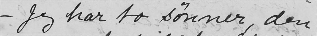- Jeg har to sønner, den
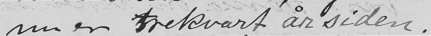nu er trekvart år siden.
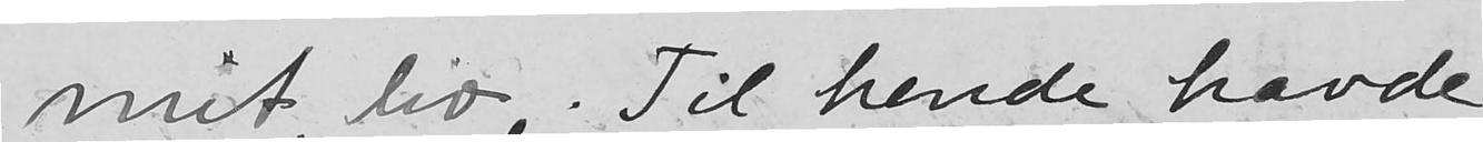mit liv. Til hende havde
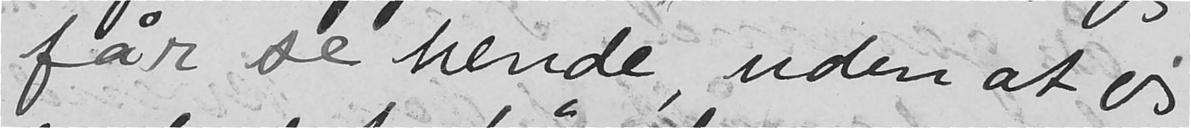får se hende, uden at er
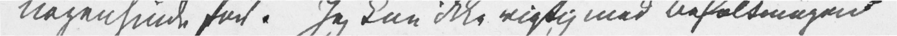nogensinde før. Jeg kan ikke rigtig med Befolkningen
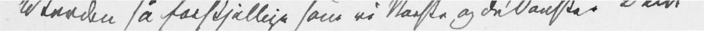Verden så forskjellige som vi Norske og de Danske. Selv
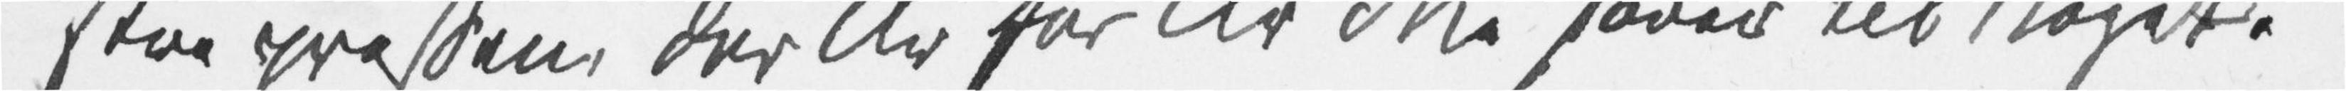strepressen, der År for År ikke fører til Noget.
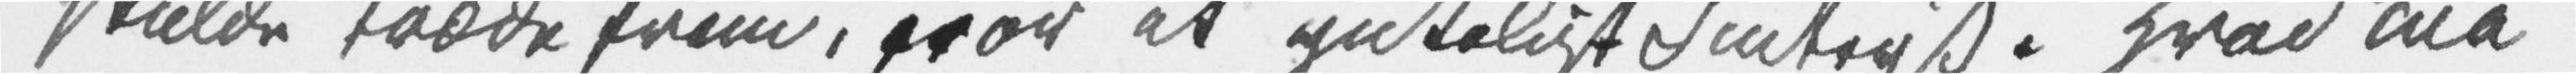skulde træde frem, gjør et ynkeligt Indtryk. Hvad må
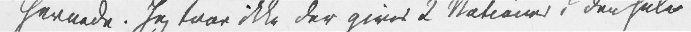hernede. Jeg tror ikke der gives 2 Nationer i den hele
